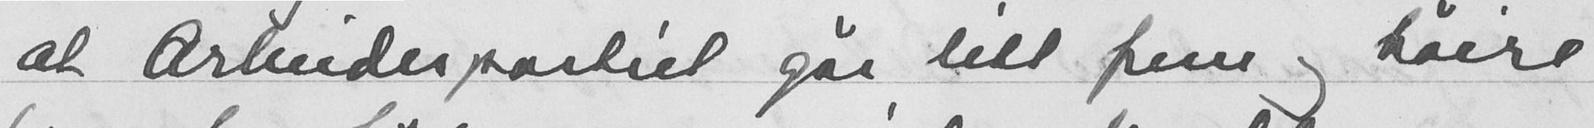at Arbeiderpartiet går litt frem og høire
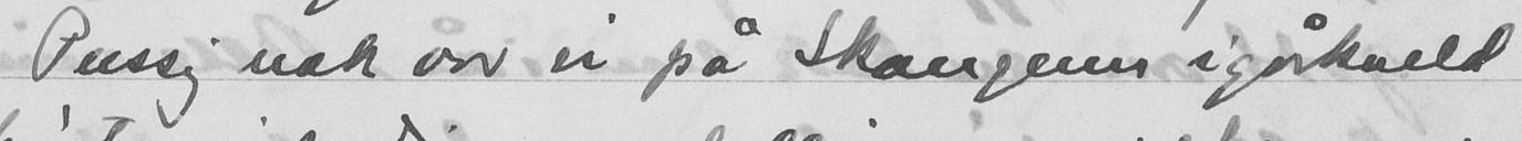Pussig nok var vi på Skaugum igårkveld
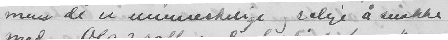men de er menneskelige og rolige å snakke
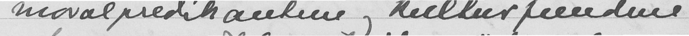moralpredikantene og kulturfiendene
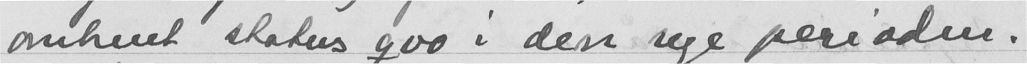omtrent status quo i den nye perioden.
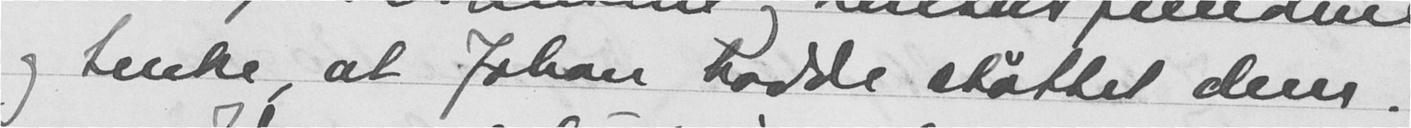og tenke at Johan hadde støttet dem.
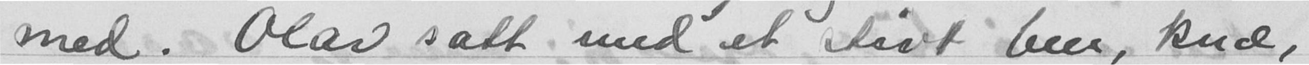med. Olav satt med et stivt ben, Rud,
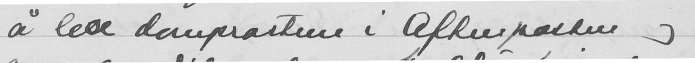å lese domprostene i Aftenposten og
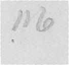116
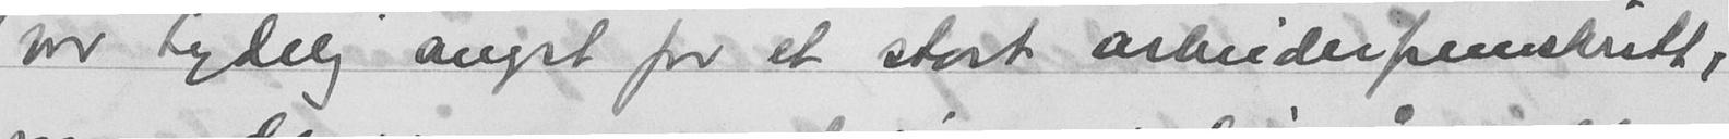var tydelig angst for et stort arbeiderfremskritt,
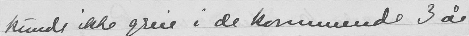kunde ikke greie i de kommende 3 år
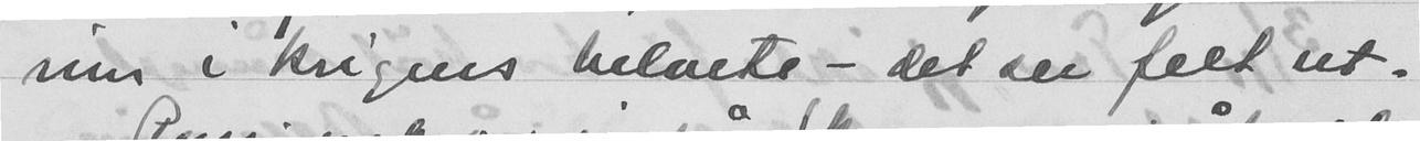inn i krigens helvete - det ser felt ut.
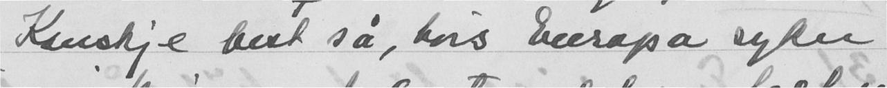Kanskje best så, hvis Europa ryker
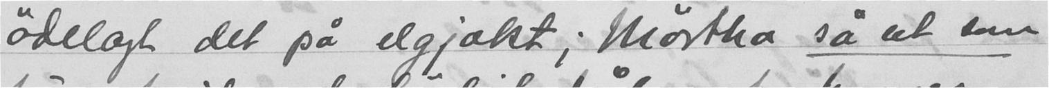ødelagt det på elgjakt; Märtha så ut som
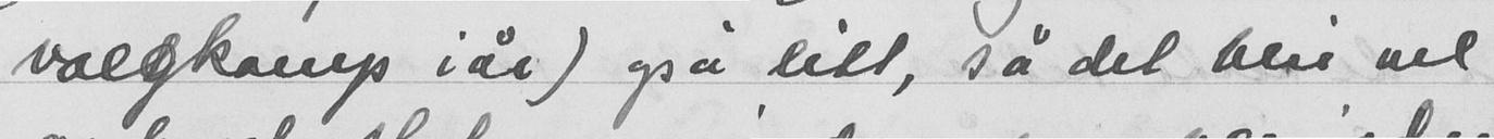valgkamp iår) også litt, så det blir vel
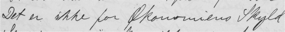Det er ikke for Økonomiens Skyld
